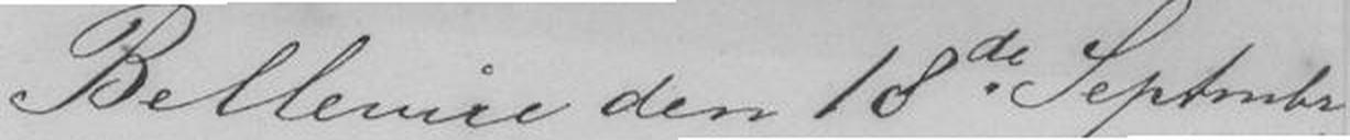Bellevue den 18de.September
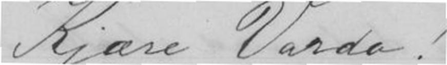Kjære Vardo!
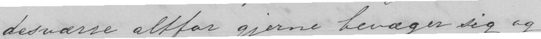desværre altfor gjerne bevæger sig og
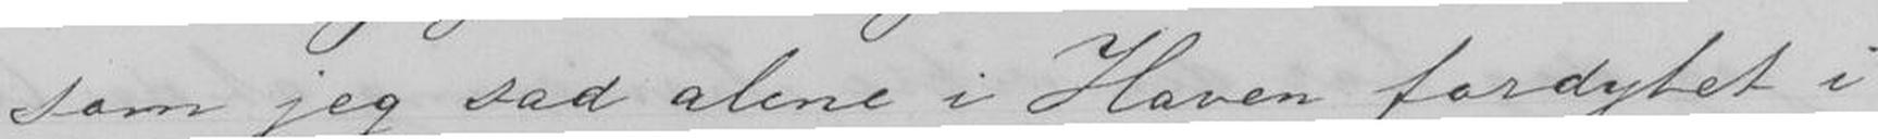som jeg sad alene i Haven fordybet i
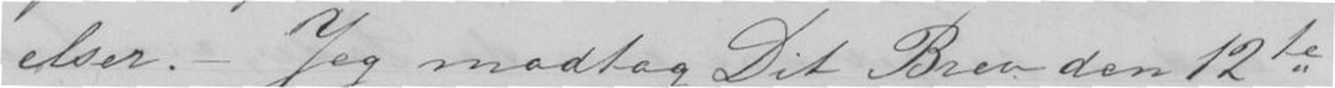elser.- Jeg modtog Dit Brev den 12te
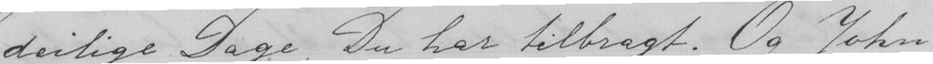deilige Dage, Du har tilbragt. Og John
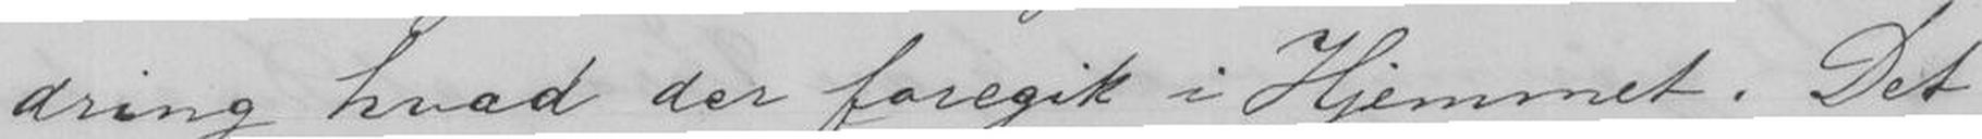dring hvad der foregik i Hjemmet. Det
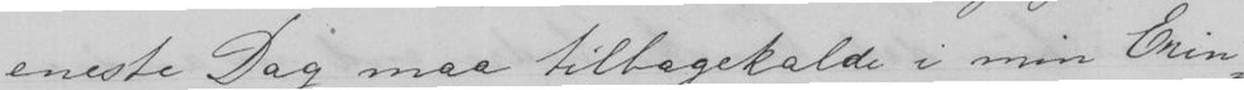eneste Dag maa tilbagekalde i min Erin-
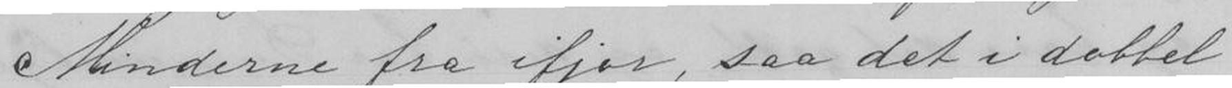Minderne fra ifjor, saa det i dobbel
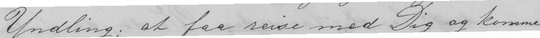Yndling; at faa reise med Dig og komme
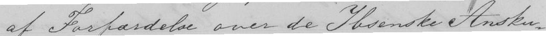af Forfærdelse over de Ibsenske Ansku-
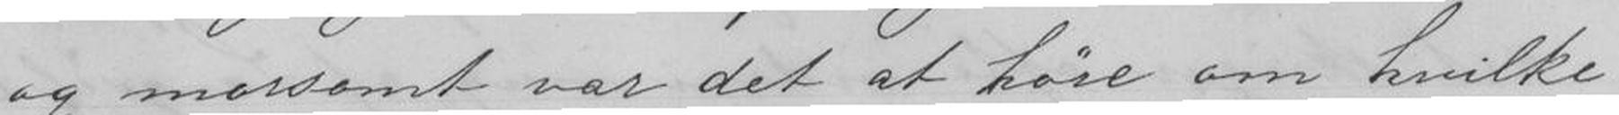og morsomt var det at høre om hvilke
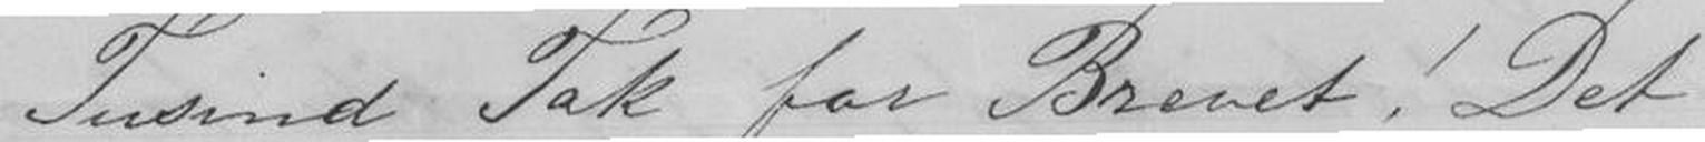Tusind Tak for Brevet! Det
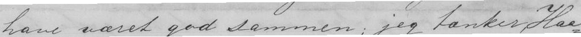have været god sammen; jeg tænker Haa-
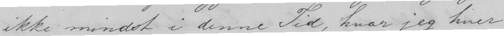ikke mindst i denne Tid, hvor jeg hver
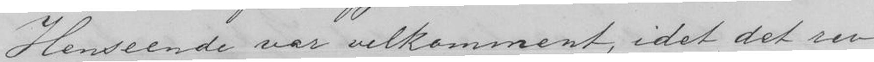Henseende var velkomment, idet det rev
